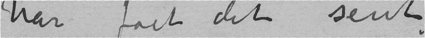har fået det sent
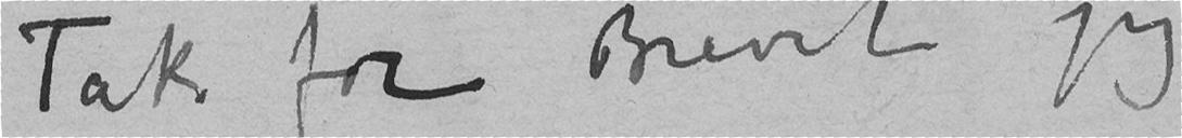Tak for Brevet jeg
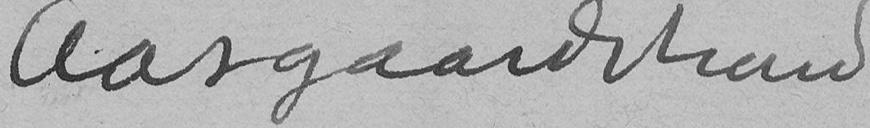Aasgaardstrand
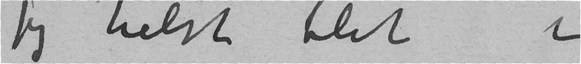jeg helst blit i
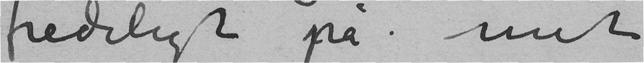fredeligt på mit
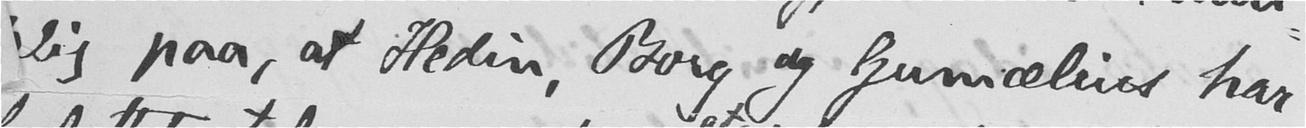lig paa, at Hedin, Borg og Gumælius har
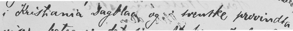i Kristiania Dagblad, og i svenske provindsa-
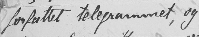forfattet telegrammet, og
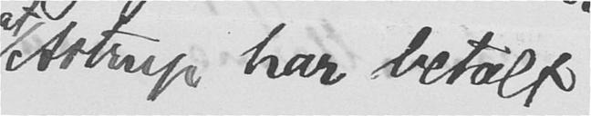Astrup har betalt
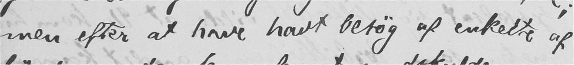men efter at have havt besøg af enkelte af
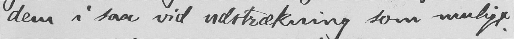dem i saa vid udstrækning som muligt.
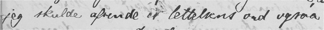jeg skulde afsende et lettelsens ord ogsaa
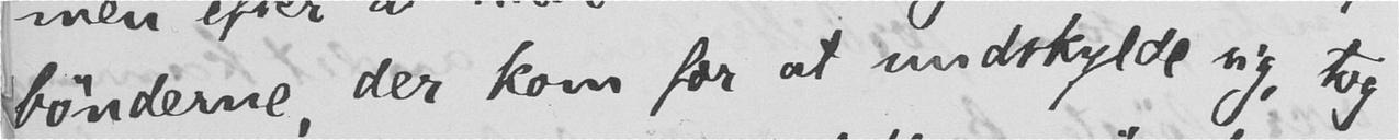bønderne, der kom for at undskylde sig, tog
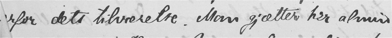rfor dets tilværelse. Man gjætter her almin-
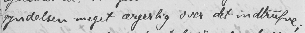gyndelsen meget ærgerlig over det indtrufne;
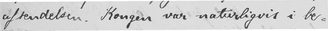afsendelsen. Kongen var naturligvis i be-
